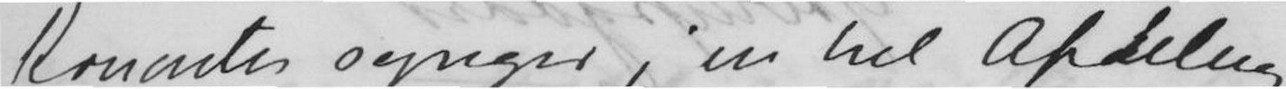Koncerter synger j en hel Afdeling
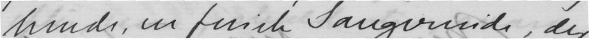hende, en furisk Sangerinde, der
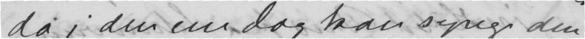da j den ene dag kan synge den
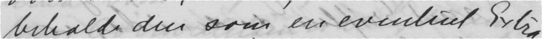beholde den som en eventuel Extra
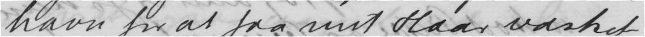havn for at faa mit Haar vasket
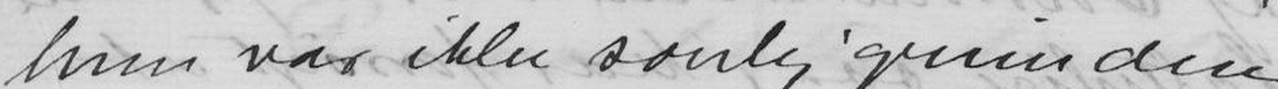hun var ikke saerlig grundne
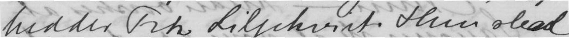hedder Frk Liljekvist. Hun skal
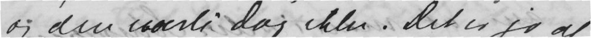og den naeste Dag ikke. Det er jo af
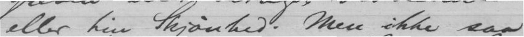eller hin Skjønhed. Men ikke saa
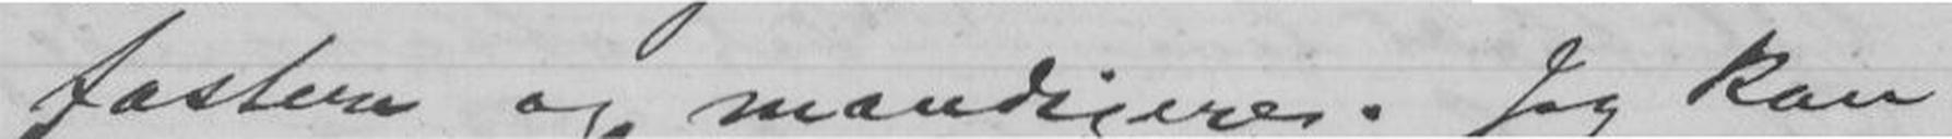fastere og mandigere. Jeg kan
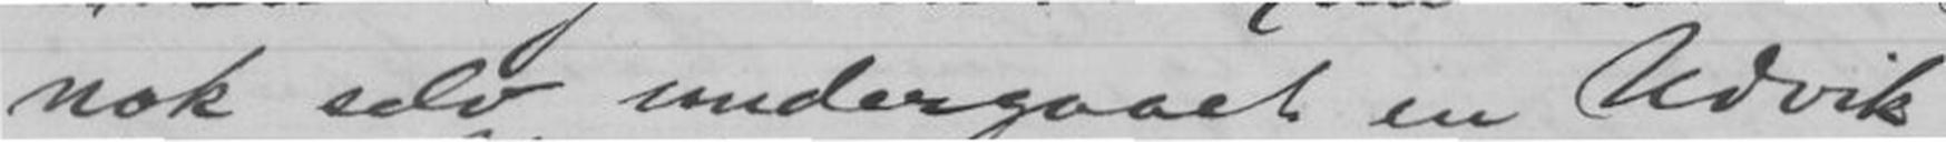nok selv undergaaet en Udvik-
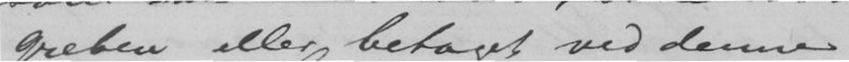greben eller betaget ved denne
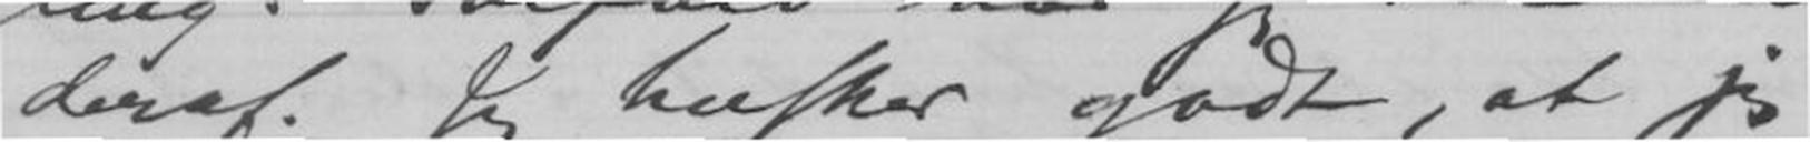deraf. Jeg husker godt, at jeg
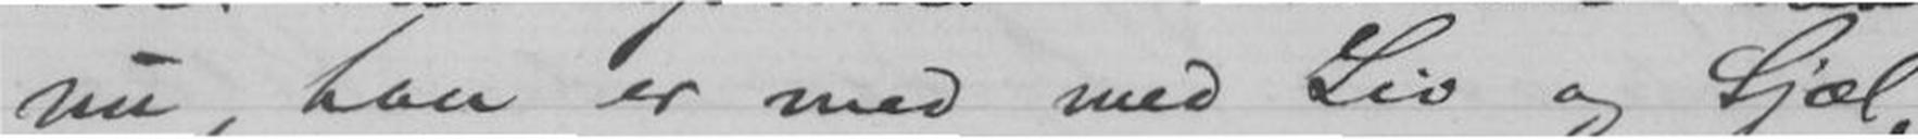nu, han er med med Liv og Sjæl,
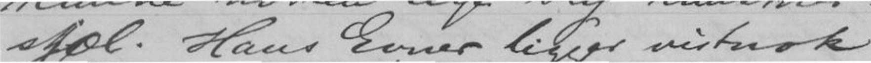sjæl. Hans Evner ligger vistnok
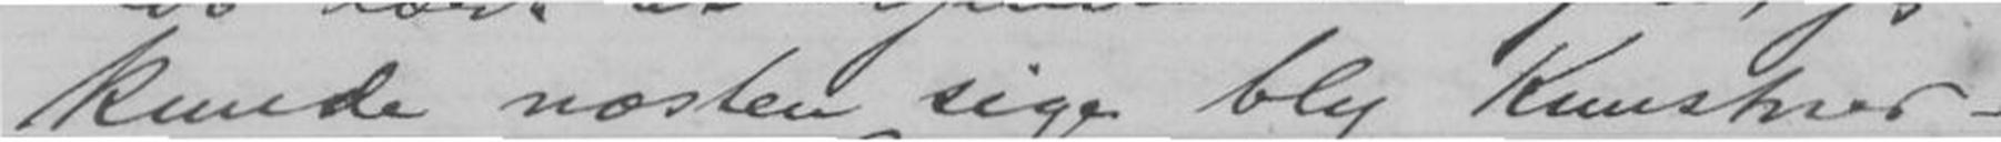kunde næsten sige bly Kunstner-
